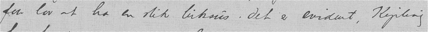faar lov at ha en slik luksus. Det er evident, Kipling
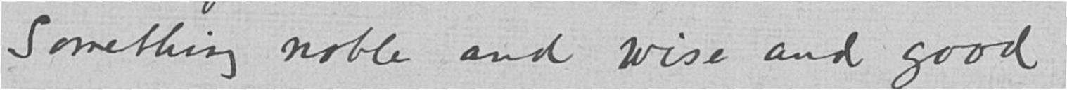Something noble and wise and good
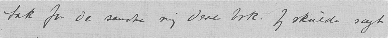tak for De sendte mig Deres bok. Jeg skulde sagt
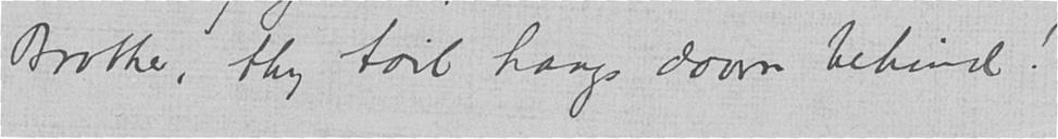Brother, thy tail hangs down behind!
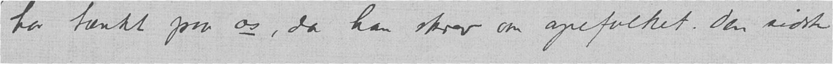har tænkt paa os, da han skrev om apefolket. Den sidste
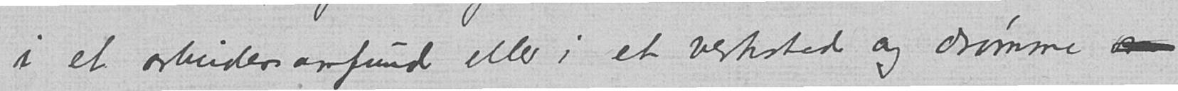i et arbeidersamfund eller i et verksted og drømme
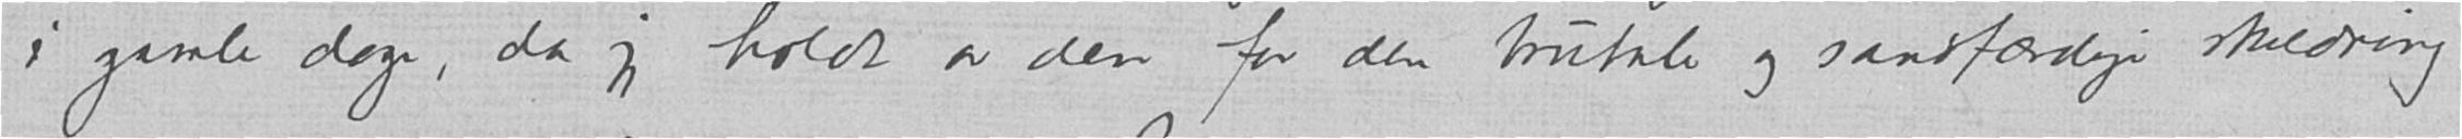i gamle dager, da jeg holdt av den for den brutale og sandfærdige skildring
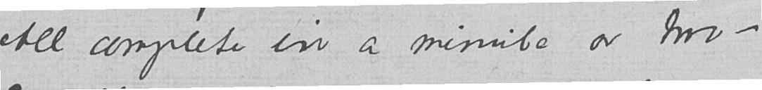All complete in a minute or two -
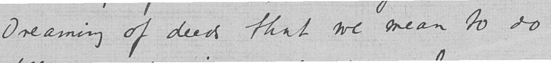Dreaming of deeds that we mean to do
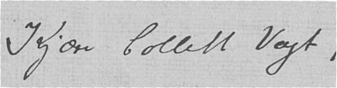Kjære Collett Vogt,
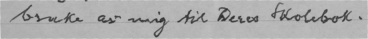bruke av mig til Deres Skolebok.
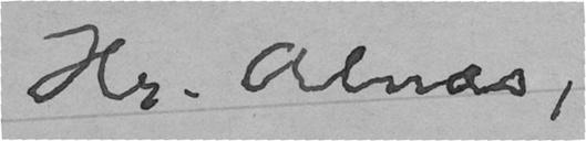Hr. Alnæs,
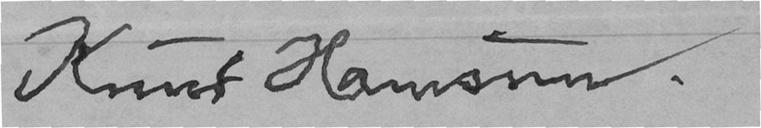Knut Hamsun.
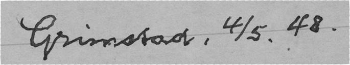Grimstad, 4/5. 48.
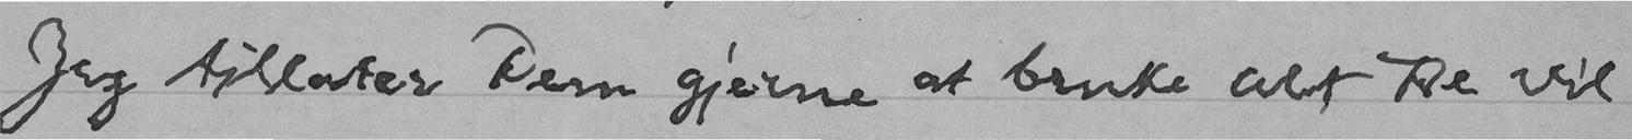Jeg tillater Dem gjerne at bruke alt De vil
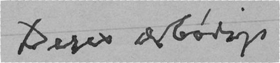Deres ærbødige
